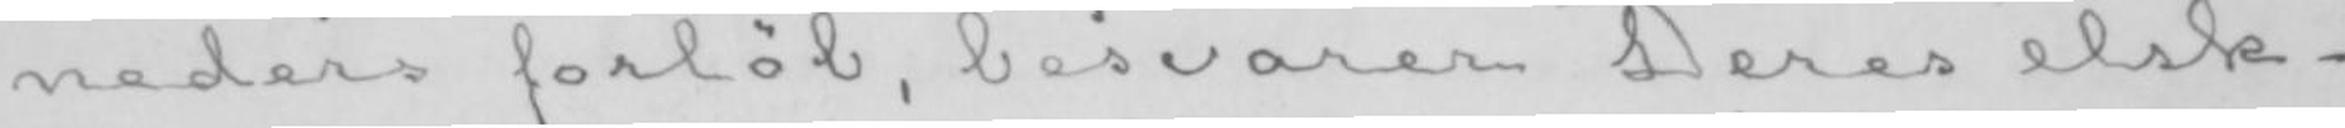neders forløb, besvarer Deres elsk-
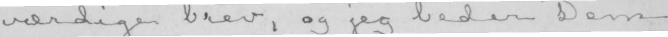værdige brev, og jeg beder Dem
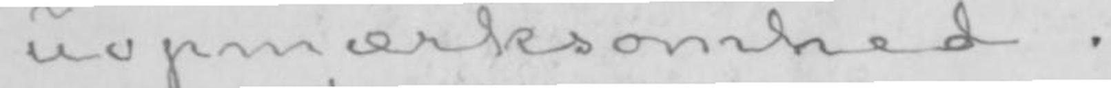uopmærksomhed.
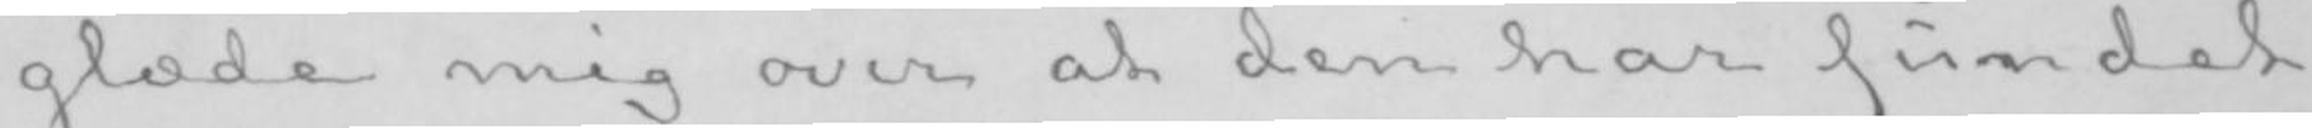glæde mig over at den har fundet
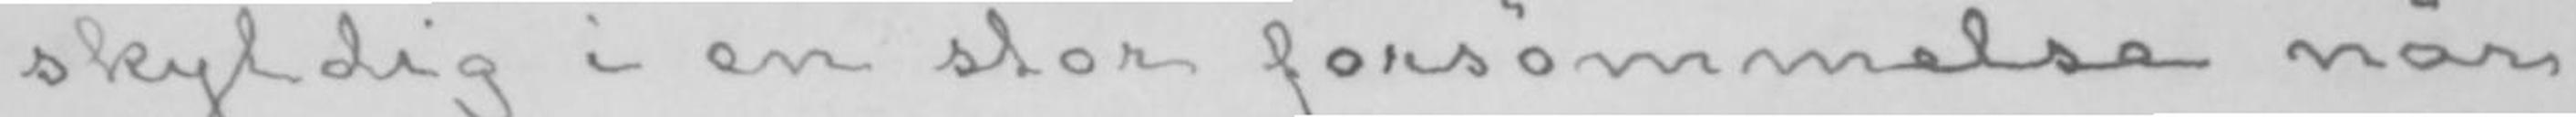skyldig i en stor forsømmelse når
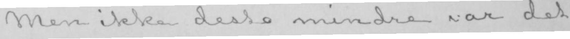Men ikke desto mindre var det
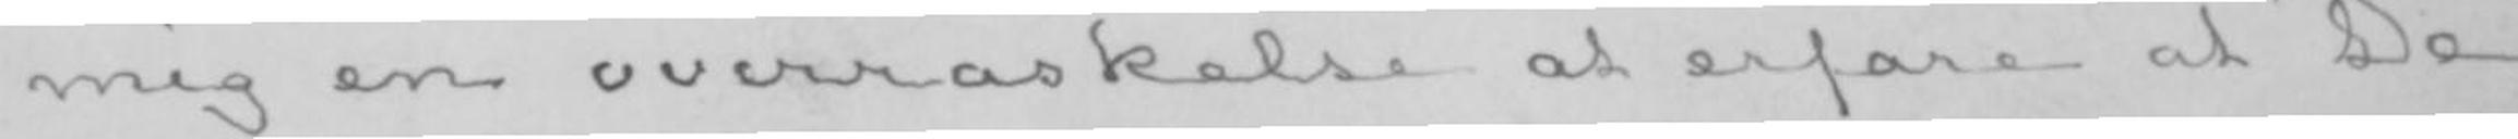mig en overraskelse at erfare at De
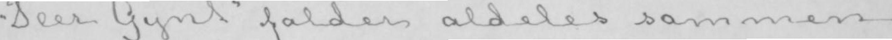«Peer Gynt» falder aldeles sammen
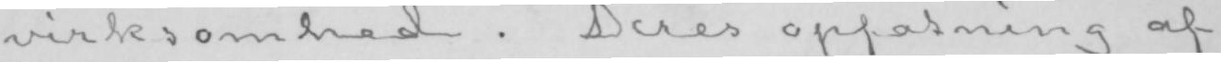virksomhed. Deres opfatning af
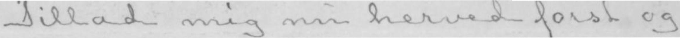Tillad mig nu herved først og
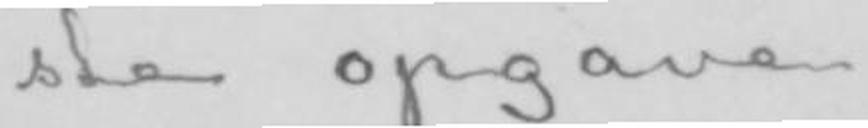ste opgave.
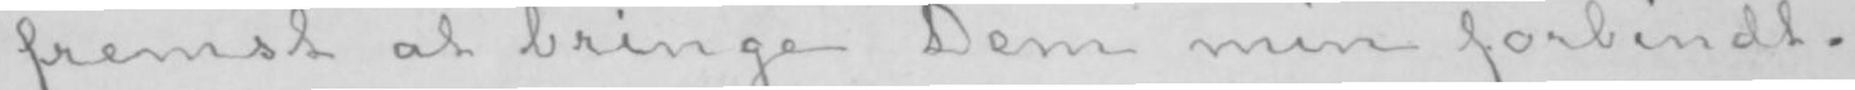fremst at bringe Dem min forbindt-
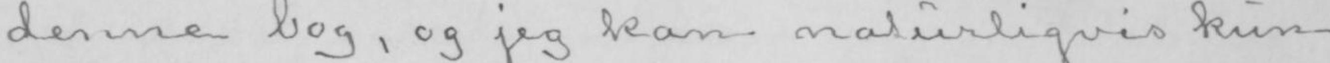denne bog, og jeg kan naturligvis kun
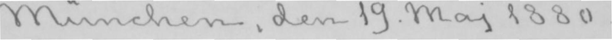München, den 19. Maj 1880.
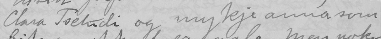Clara Tschidi og mykje anna som
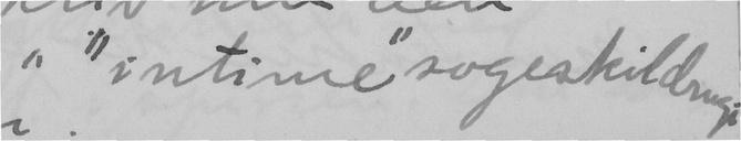"intime" sogeskildringi
